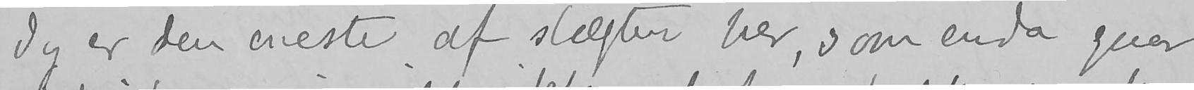Jeg er den eneste af slægten her, som enda gaar
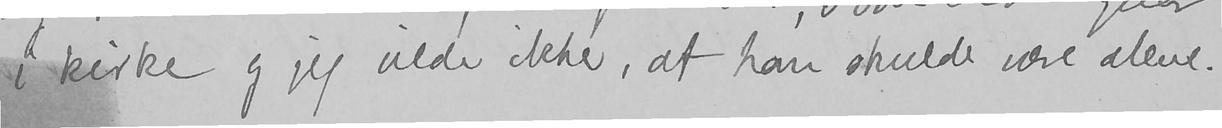i kirke og jeg vilde ikke, at han skulde være alene.
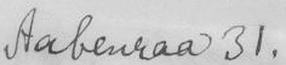Aabenraa 31.
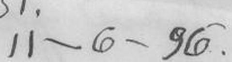11-6-96.
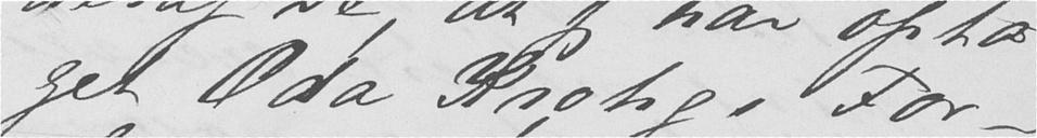get Oda Krohgs For-
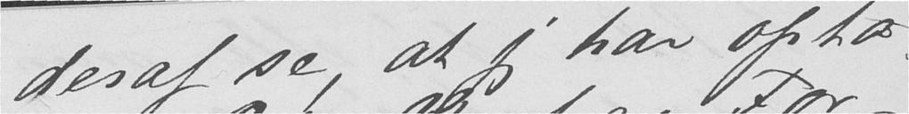deraf se, at jeg har opta-
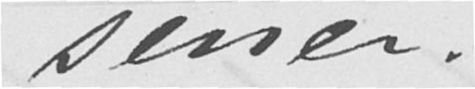sener.
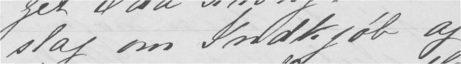slag om Indkjøb af
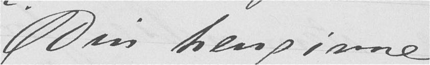Din hengivne
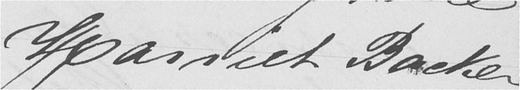Harriet Backer
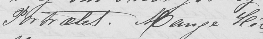Portrætet. Mange Hil-
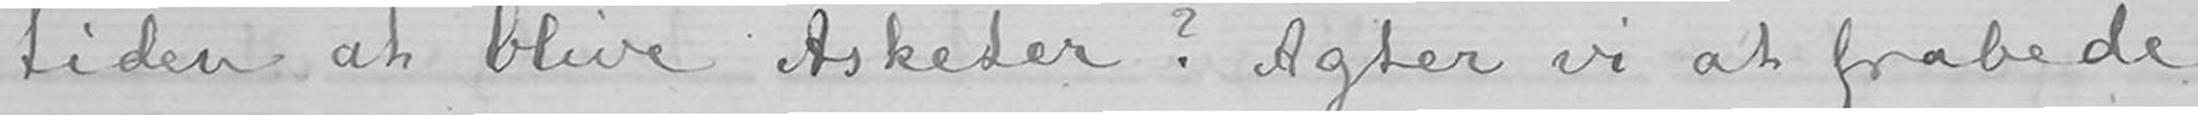tiden at blive Asketer? Agter vi at frabede
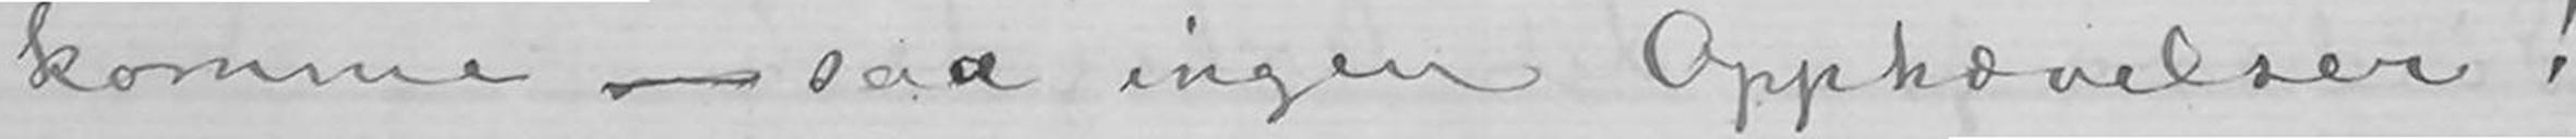komme- saa ingen Opphævelser!
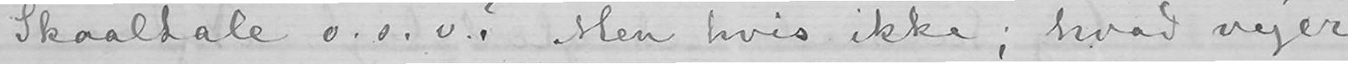Skaaltale o. s. v.? Men hvis ikke; hvad vejer
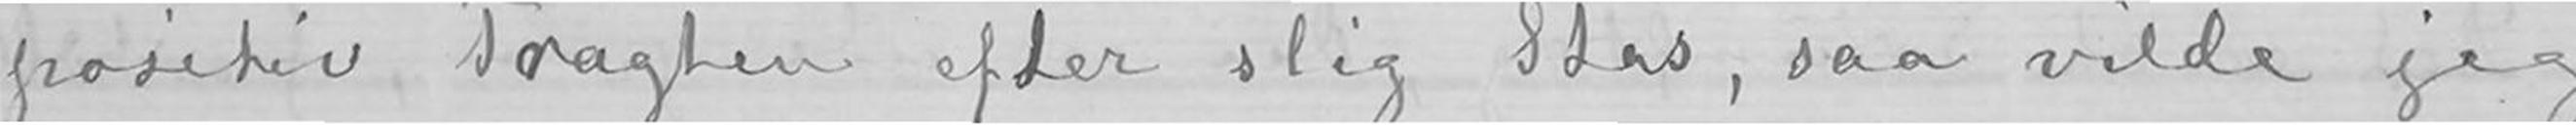positiv Tragten efter slig Stas, saa vilde jeg
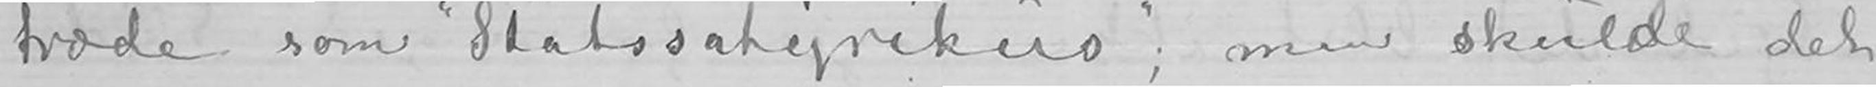træde som «Statssatyrikus»; men skulde det
